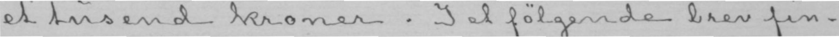et tusend kroner. I et følgende brev fin-
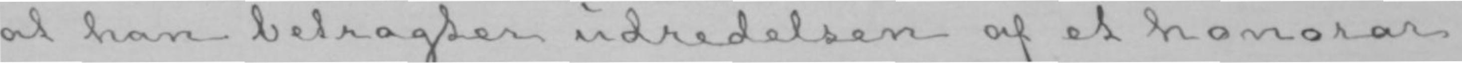at han betragter udredelsen af et honorar
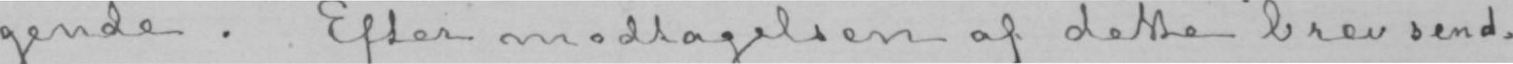gende. Efter modtagelsen af dette brev send-
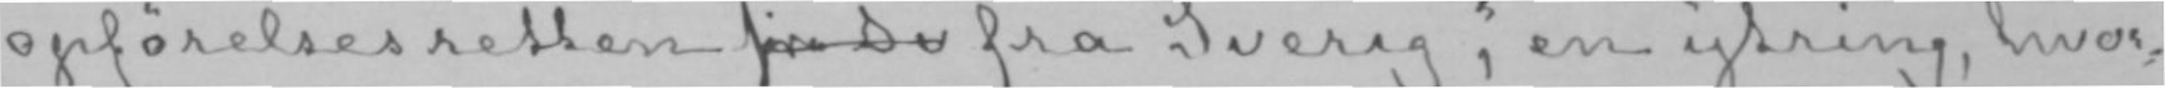opførelsesretten fr Sv fra Sverig,» en ytring, hvor-
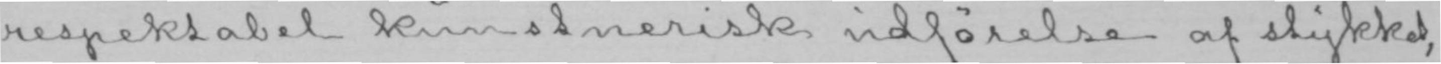respektabel kunstnerisk udførelse af stykket,
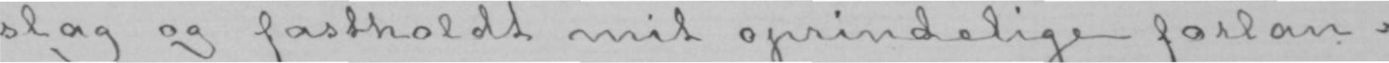slag og fastholdt mit oprindelige forlan-
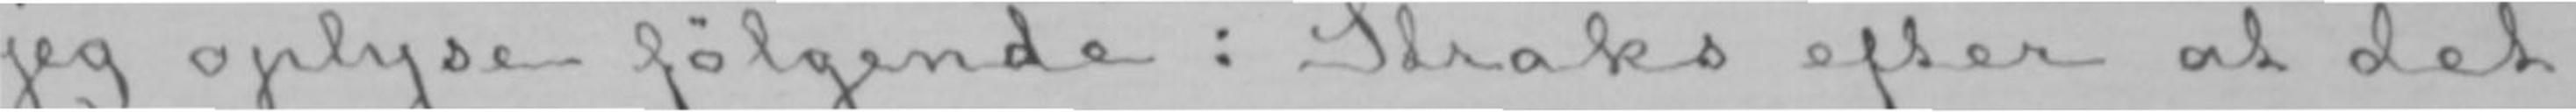jeg oplyse følgende: Straks efter at det
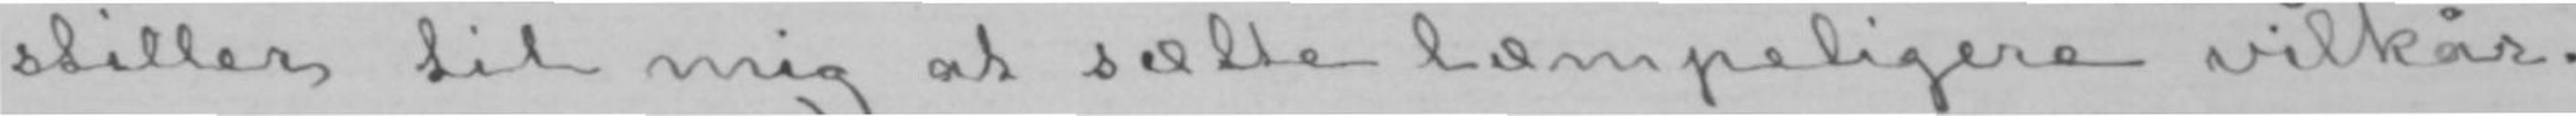stiller til mig at sætte læmpeligere vilkår.
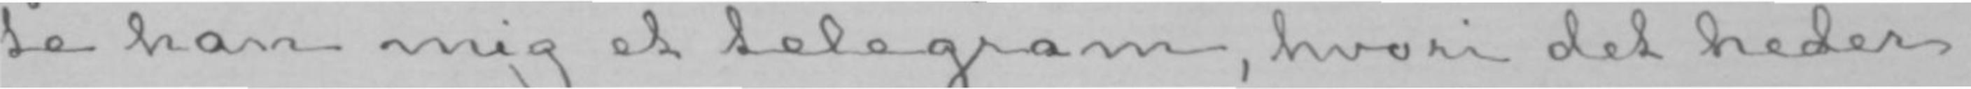te han mig et telegram, hvori det heder
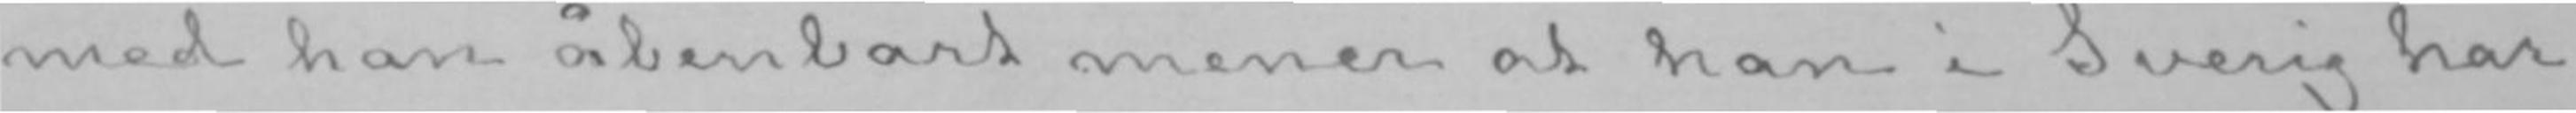med han åbenbart mener at han i Sverig har
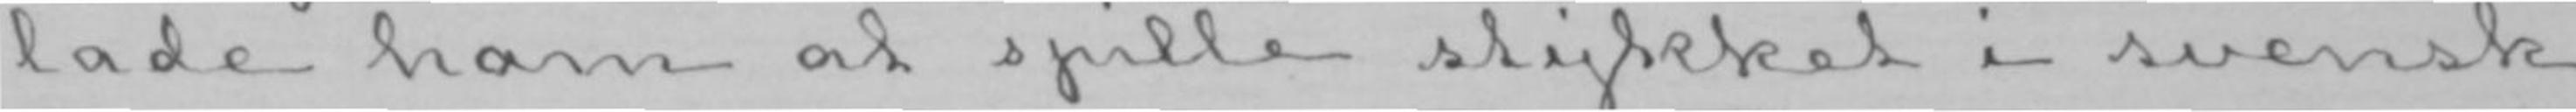lade ham at spille stykket i svensk
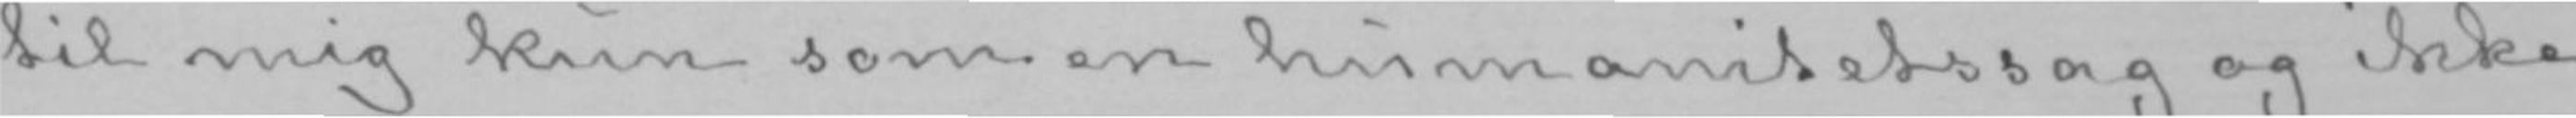til mig kun som en humanitetssag og ikke
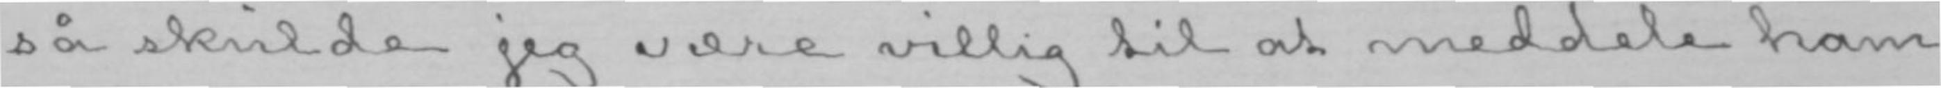så skulde jeg være villig til at meddele ham
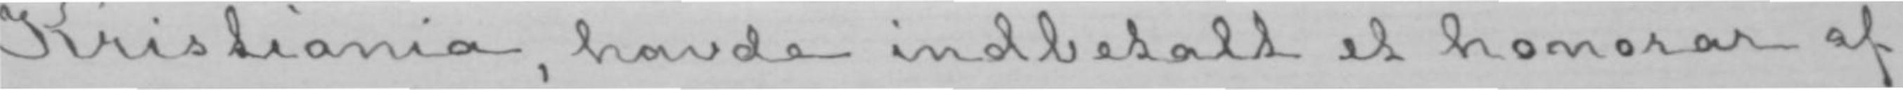Kristiania, havde indbetalt et honorar af
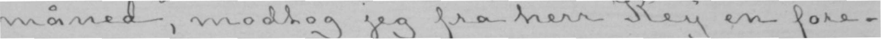måned, modtog jeg fra herr Key en fore-
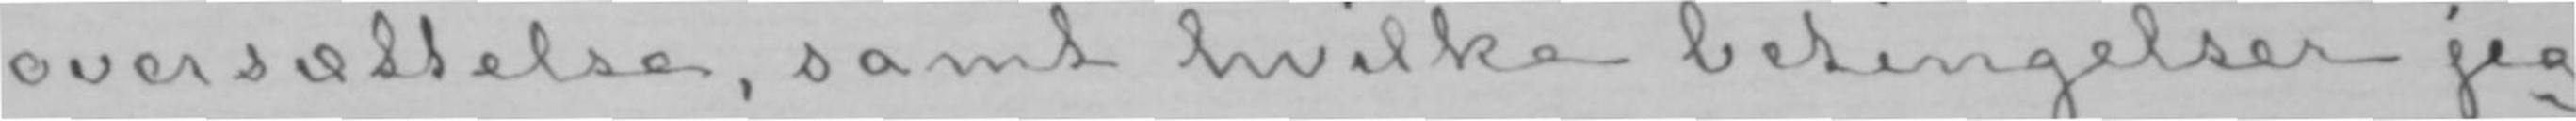oversættelse, samt hvilke betingelser jeg
